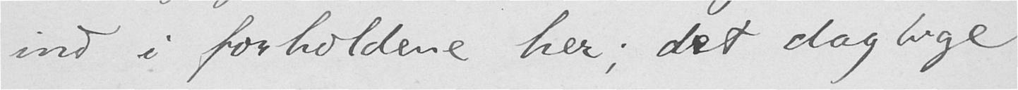ind i forholdene her; det daglige
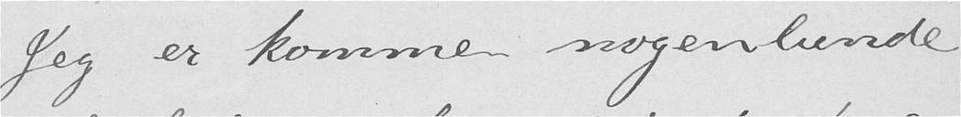Jeg er kommen nogenlunde
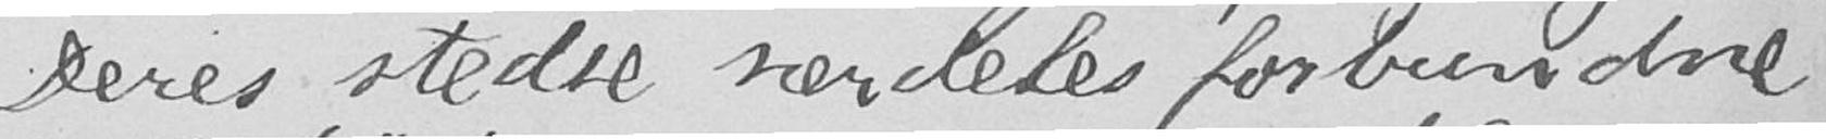Deres stedse særdeles forbundne
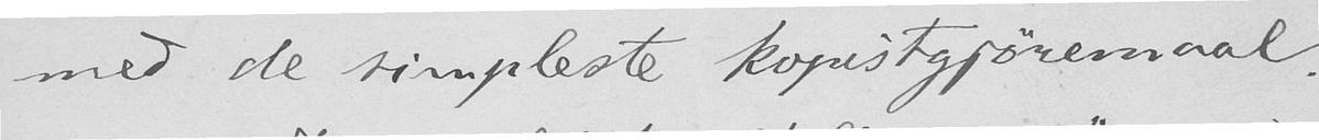med de simpleste kopistgjøremaal.
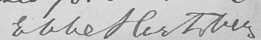Ebbe Hertzberg
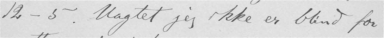12-5. Uagtet jeg ikke er blind for
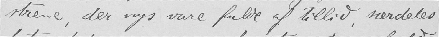strene, der nys vare fulde af tillid, særdeles
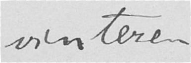vinteren.
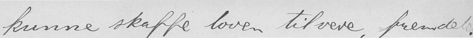kunne skaffe loven tilveie, fremdeles
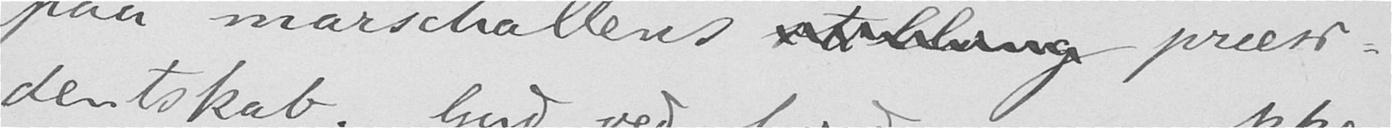paa marschallens  præsi-
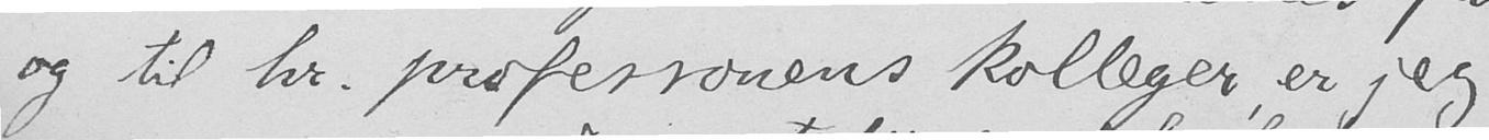og til hr. professorens kolleger, er jeg
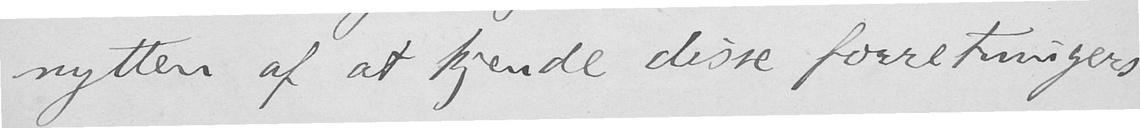nytten af at kjende disse forretningers
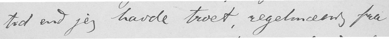tid end jeg havde troet, regelmæssig fra
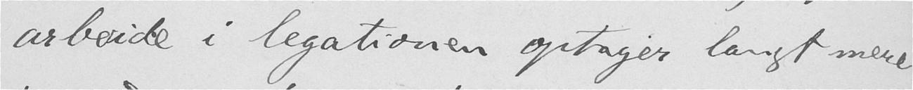arbeide i legationen optager langt mere
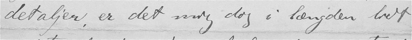detaljer, er det mig dog i længden lidt
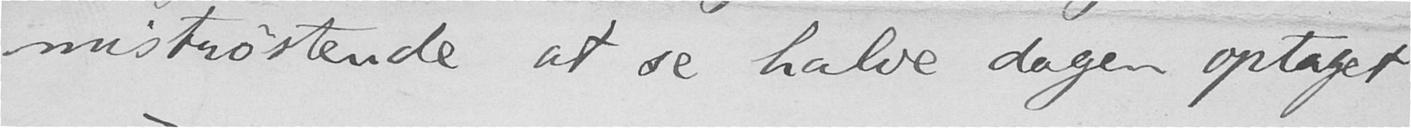mistrøstende at se halve dagen optaget
